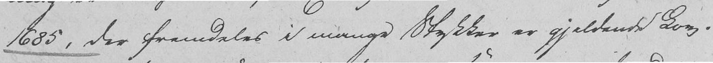1685, der fremdeles i mange Stykker er gjeldende Lov.
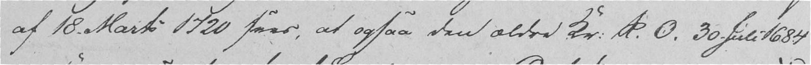af 18 Marti 1720 sees, at ogsaa den ældre Kr. R. O. 30. Juli 1684
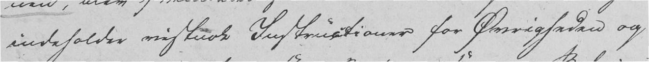indeholder vistnok Instructioner for Øvrigheden og
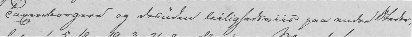Taxereborgere og desuden leilighedsviis paa andre Steder,
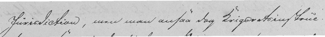Jurisdiction, men man ansaa dog Krigsretsinstruc-
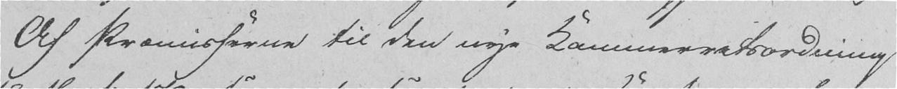Af Præmisserne til den nye Kammerretsordning
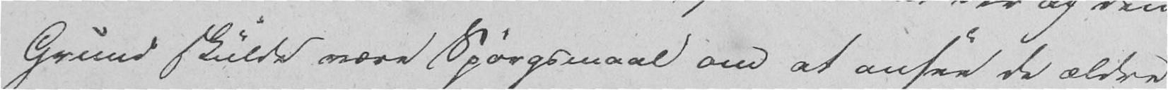Grund skulde være Spørgsmaal om at ansee de ældre
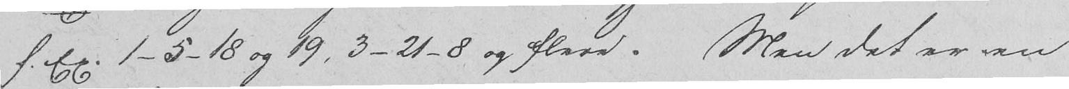f. Ex. 1-5-18 og 19, 3-21-8 og flere. Men det er en
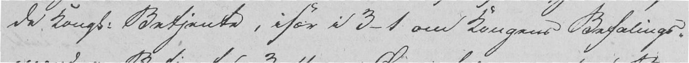de Kongl. Betjente, især i 3-1 om Kongens Befalings-
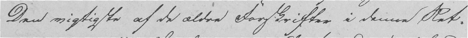Den vigtigste af de ældre Forskrifter i denne Ret-
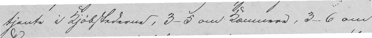tjente i Kjøbstederne, 3-5 om Kæmnere, 3-6 om
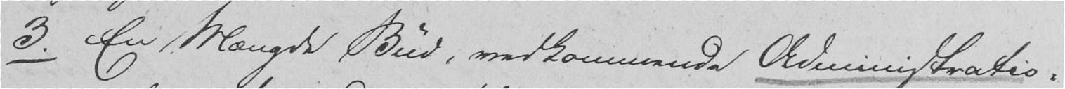3. En Mængde Bud, vedkommende Administratio-
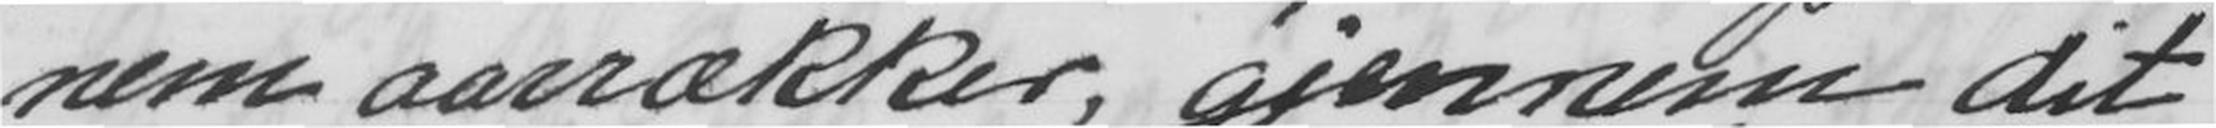nem aarækker, gjennem dit
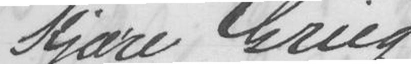Kjære Grieg
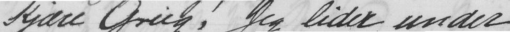Kjære Grieg! Jeg lider under
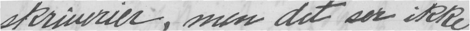skriverier, men det ser ikke
